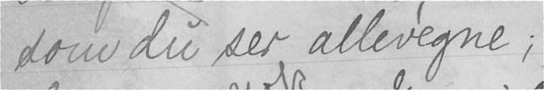som du ser allevegne;
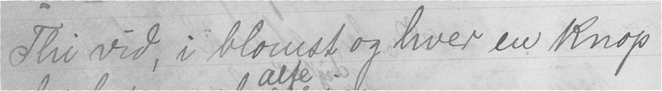Thi vid, i blomst og hver en knop
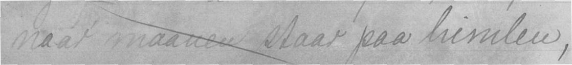naar maanen staar paa himlen,
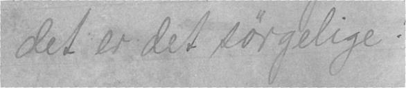det er det sørgelige!
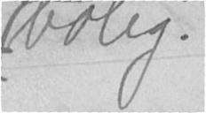bolig.
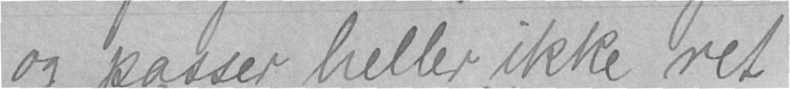og passer heller ikke ret
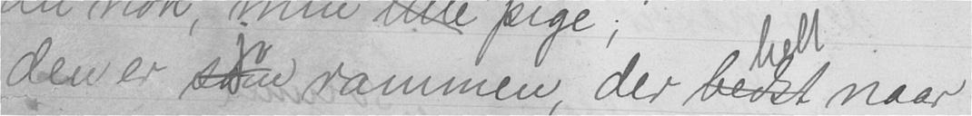den er jo rammen, der hell naar
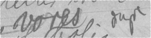vores dyre
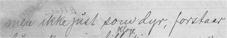men ikke just som dyr, forstaar
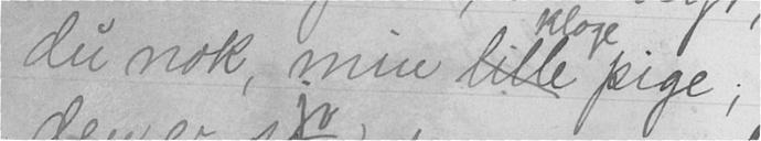du nok, min kloge pige;
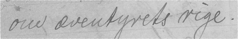om eventyrets rige.
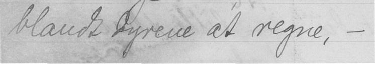blandt dyrene at regne, -
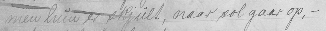men hun er skjult, naar sol gaar op, -
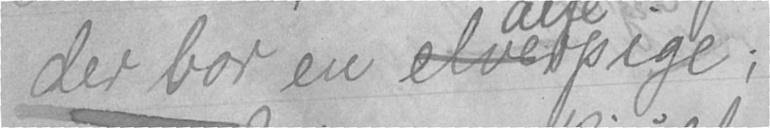der bor en alfepige;
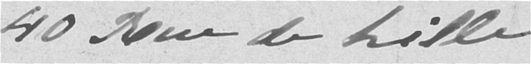40 Rue de Lille
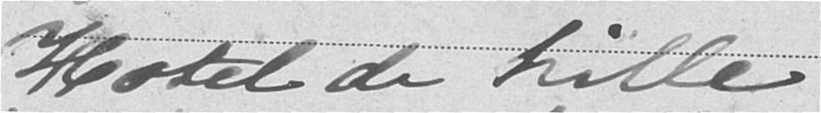Hotel de Lille
